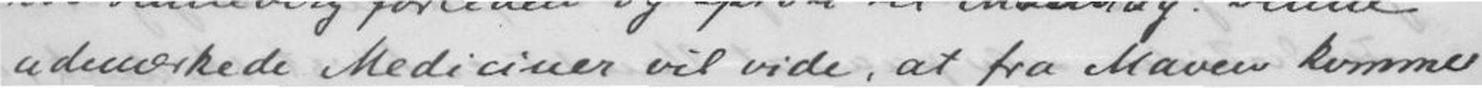udemærkede Mediciner vil vide, at fra Maven komme
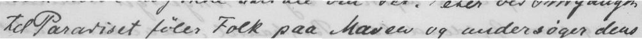til Paradiset føler Folk paa Maven og undersøger dens
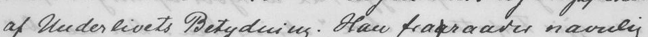af Underlivets Betydning. Han fraraader navnlig
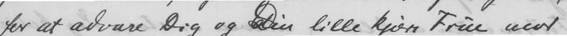for at advare Dig og Din lille kjære Frue mod -
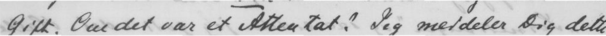Gift. Om det var et Attentat? Jeg meddeler Dig dette
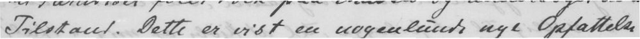Tilstand. Dette er vilst en nogenlunde nye Opfattelse
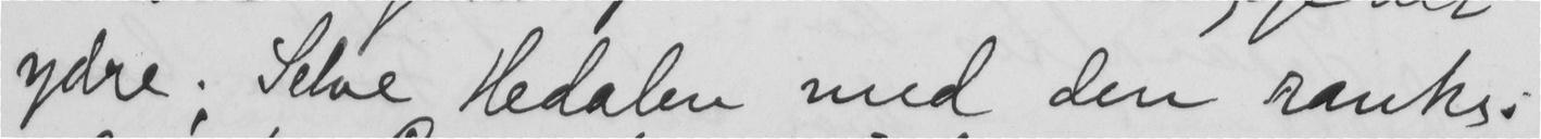ydre. Selve Hedalen med den ranke
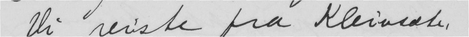Vi reiste fra Kleivsæter
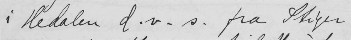i Hedalen d. v. s. fra Stiger
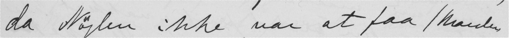da Nøglen ikke var at faa (Manden
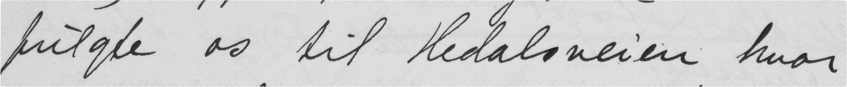fulgte os til Hedalsveien hvor
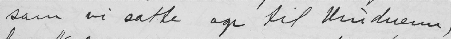som vi satte op til Vinduene,
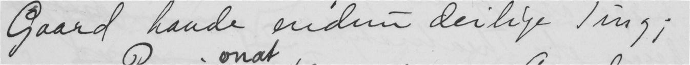Gaard havde endnu deilige Ting;
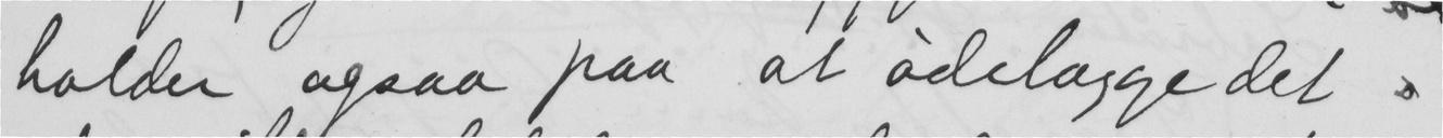holder ogsaa paa at ødelægge det;
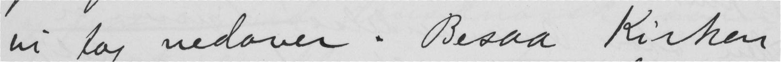vi tog nedover. Besaa Kirken
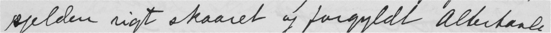sjelden rigt skaaret og forgyldt Altertavle
